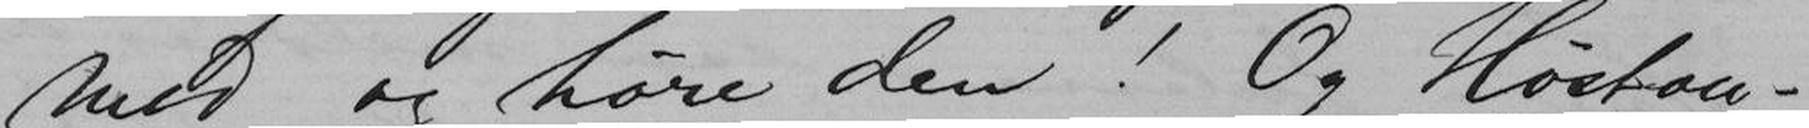med og høre den! Og Høstou-
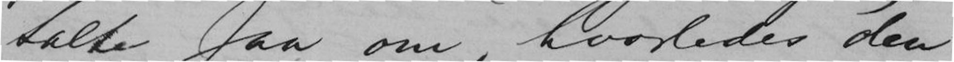talte saa om, hvorledes den
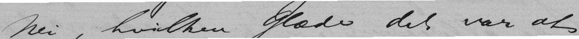Nei, hvilken Glæde det var at
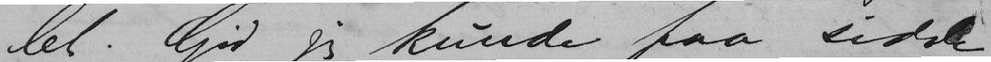let. Gid jeg kunde faa sidde
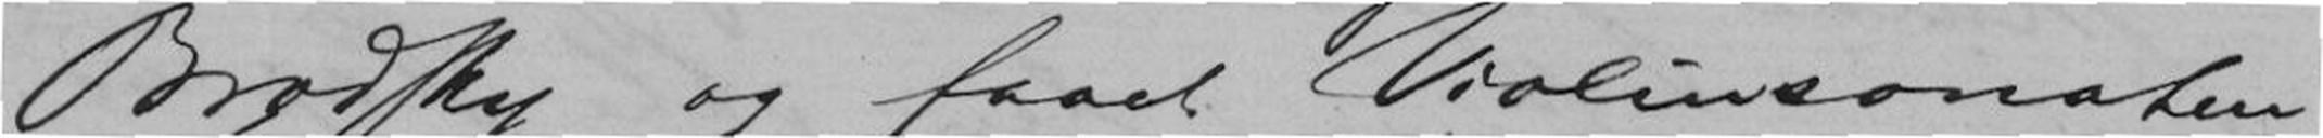Brodsky og faaet Violinsonaten
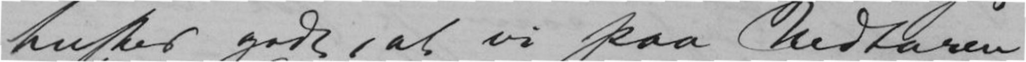husker godt, at vi paa Nedturen
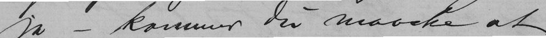ja, - kommer du maaske at
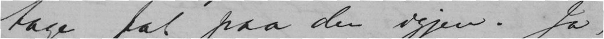tage fat paa den igjen. Ja,
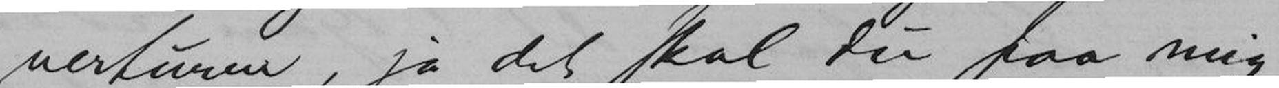verturen, ja det skal du faa mig
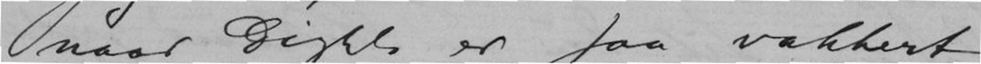naar Digtet er saa vakkert. -
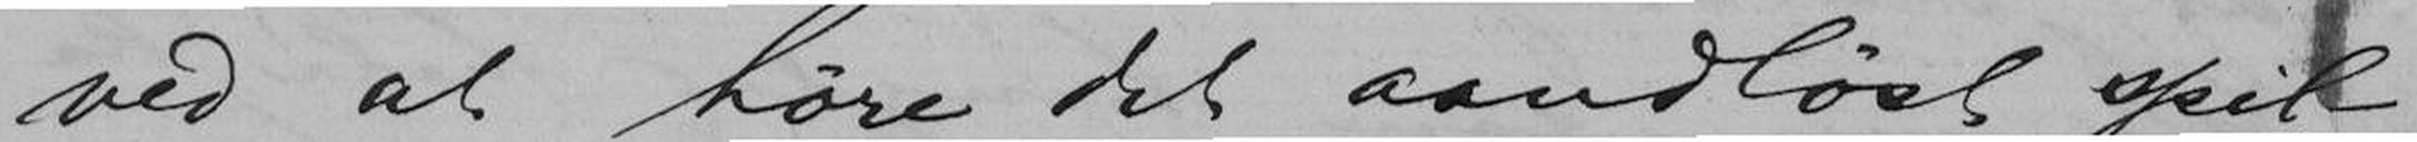ved at høre det aandløst spil-
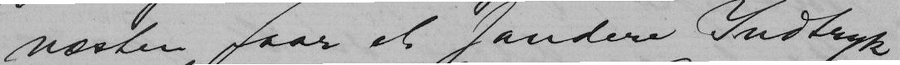næsten faar et sandere Indtryk
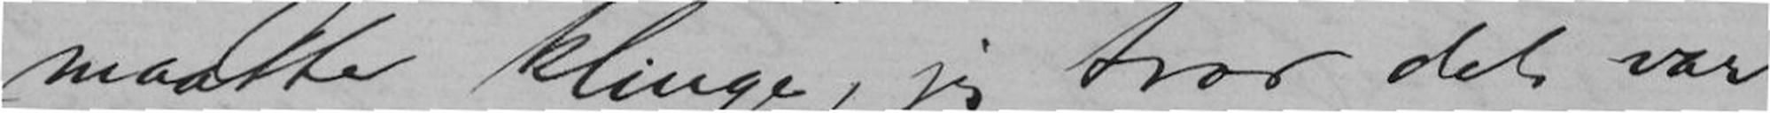maatte klinge, jeg tror det var
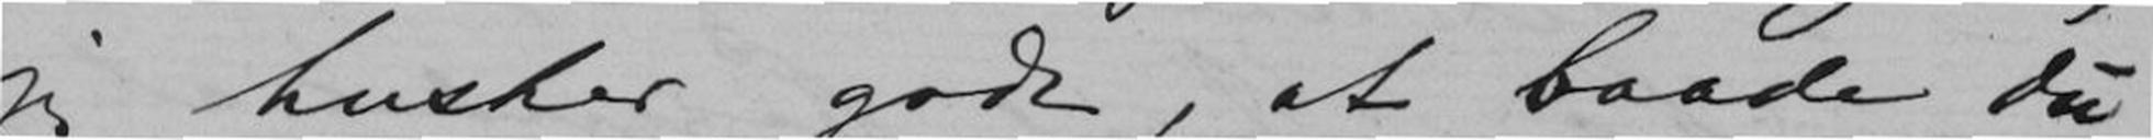jeg husker godt, at baade du
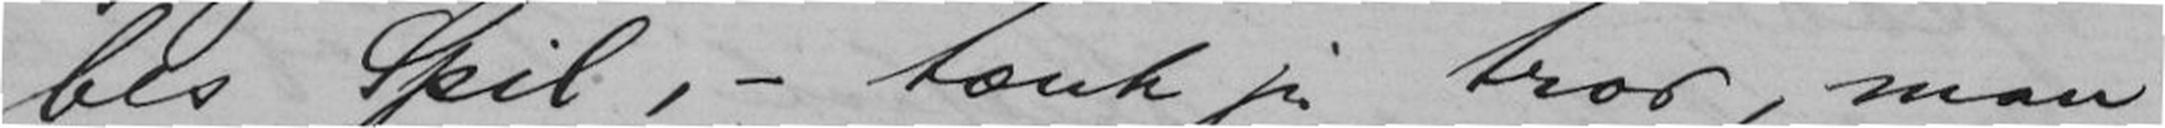bes Spil, - tænk jeg tror, man
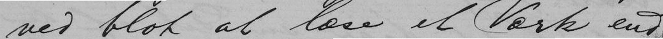ved blot at læse et Værk end
